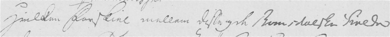Hvilken forskiel mellem disse og de Romsdalske Fielde
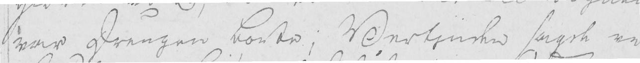Svar Drengen borte; Vertinden sagde vi
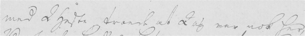med 2 Heste troede at 2 og var nok her,
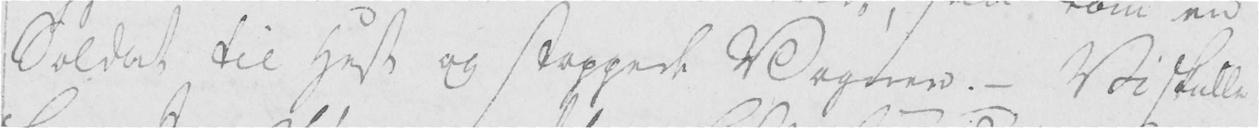Soldat til Hest og stoppede Vognen. - Vi skulle
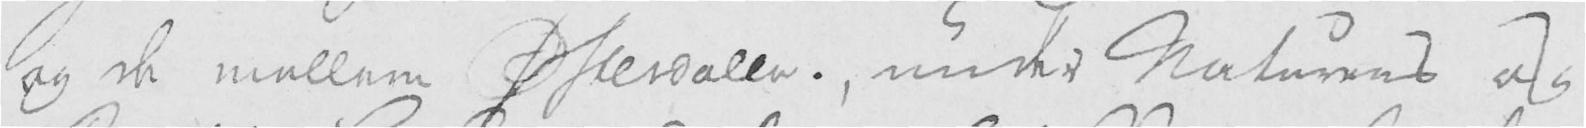og de mellem Østerdalen., under Naturens og
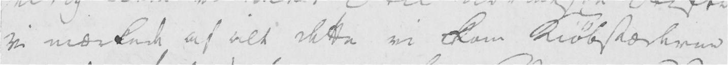vi mærkede af alt dette vi kom Kiøbstæderne
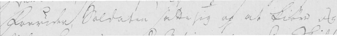Forrider, Soldaten satte sig op at kiøre og
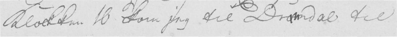Klokken 10 kom jeg til Dramdal til
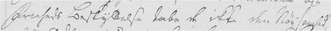Frieheds Beskyttelse tabe de ikke den Nøysomhed,
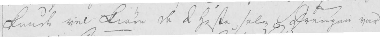kunde vel kiøre de 2 Heste selv; Drengen var
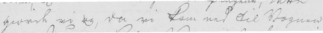giorde vi og, da vi kom ned til Vognen
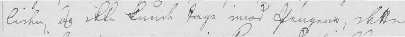liden, og ikke kunde tage mod Pengene, dette
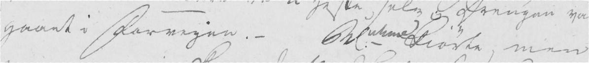gaaet i Forveyen. - Bluhme kiørte, men
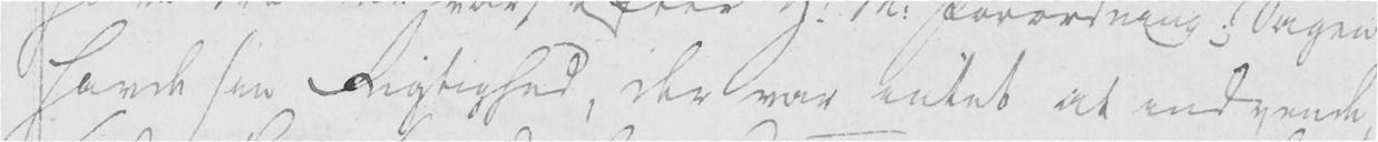havde sin Rigtighed, der var intet at indvende,
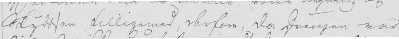Skydsen tilligemed, derfore, da Drengen var
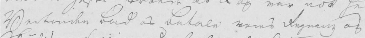Vertinden bad os betale vores Regning og
